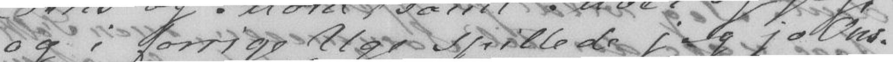og i forrige Uge spillede jeg jo Ons-
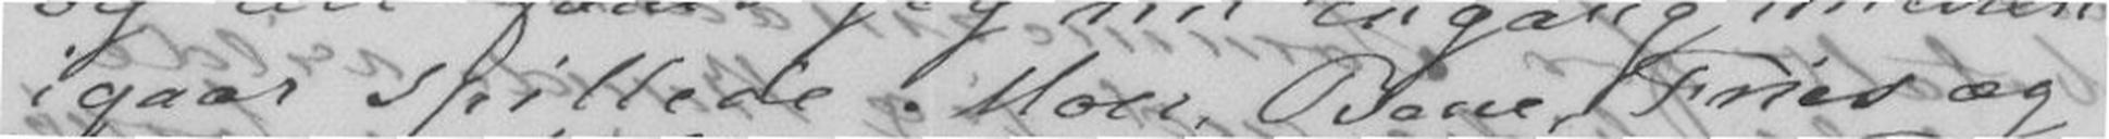igaar spillede Moer, Bene, Fries og
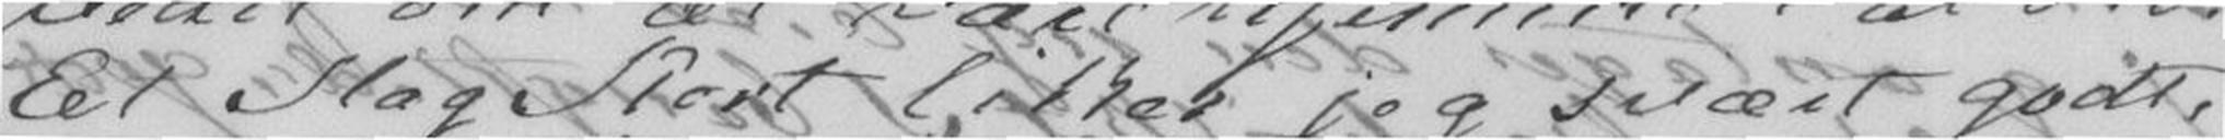Et Slag Kort liker jeg svært godt,
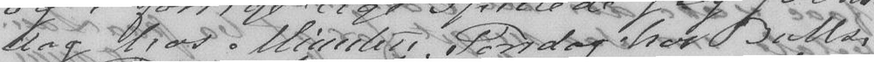dag hos Münsters. Torsdag hos Bulls,
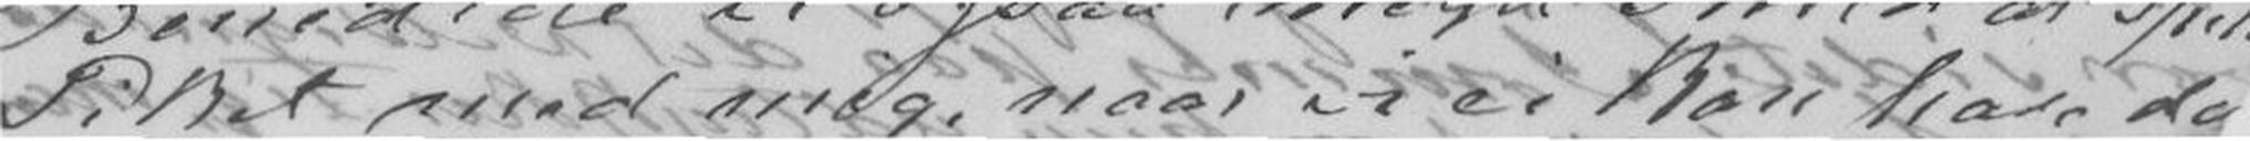Piket med mig, naar vi ei kan have de
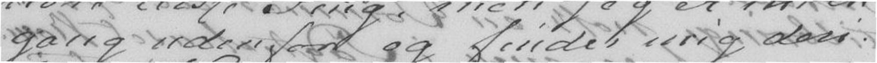gang udenfor og finder mig deri.
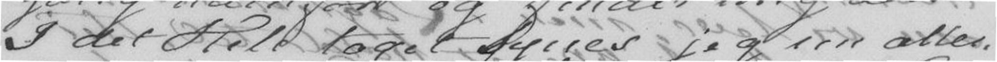I det Hele taget synes jeg nu aller-
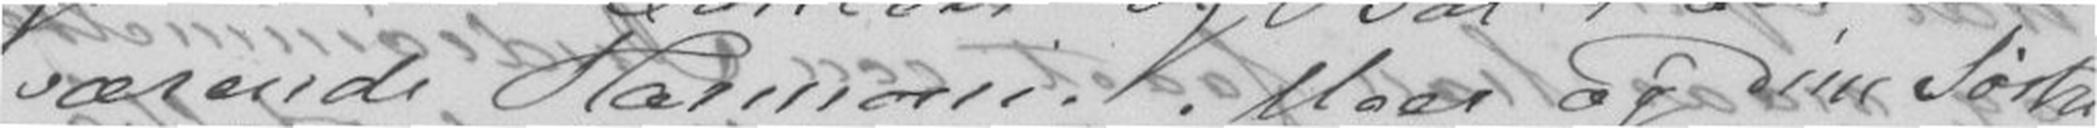værende Harmoni. Moer og Dine Søstre
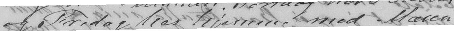og Fredag her hjemme med Maren
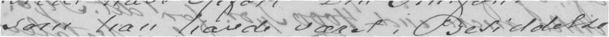som han havde været i Besiddelse
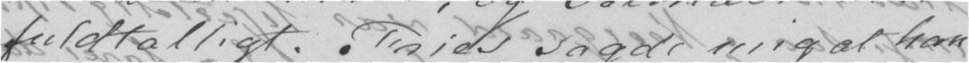fuldtalligt. Fries sagde mig at han
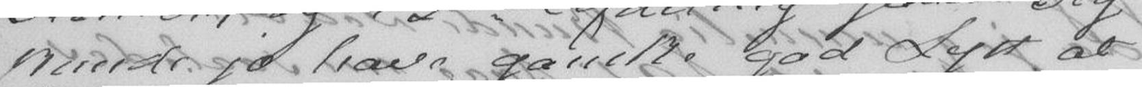kunde jo have ganske god Lyst at
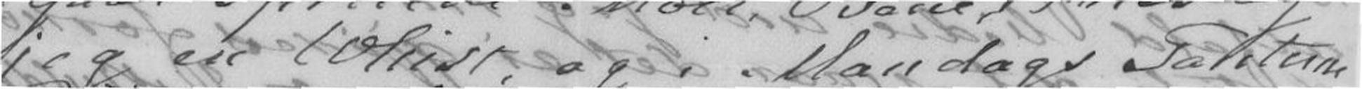jeg en Whist, og i Mandags Tanterne
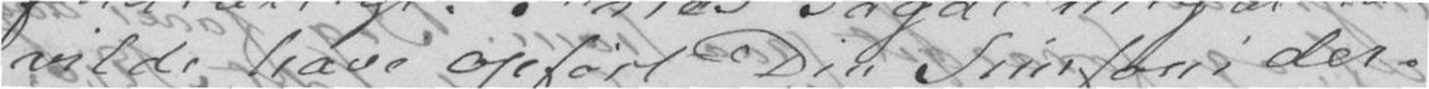vilde have opført Din Simfoni der-
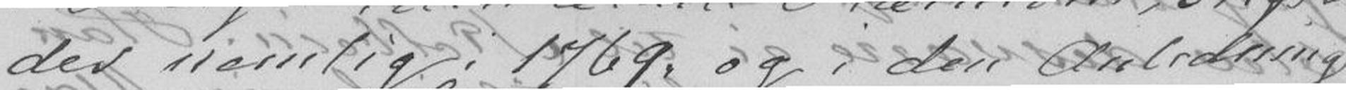des nemlig i 1769, og i den Anledning
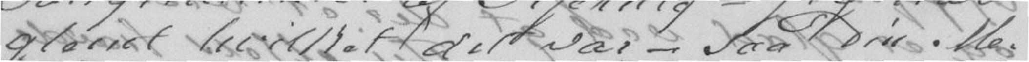glamt hvilket det var - saa Din Me-
